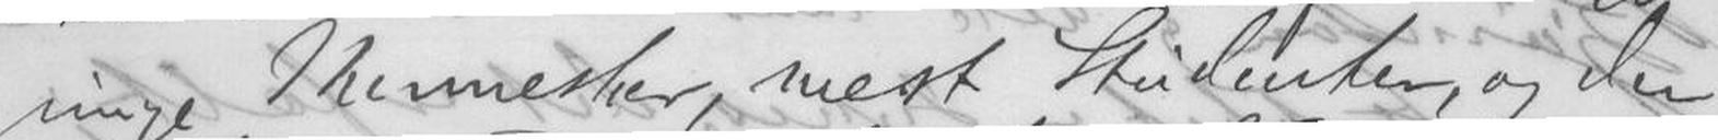unge Mennesker, mest Studenter, og der
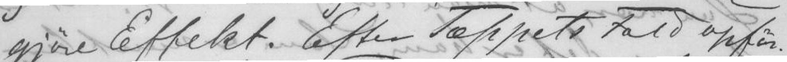gjøre Effekt. Efter Tæppets Fald opfør-
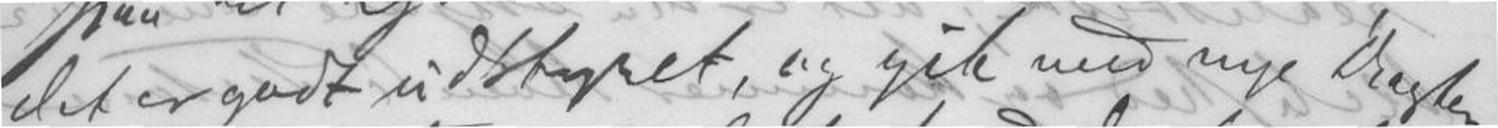det er godt udstyret, og gik med nye Dragter
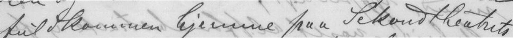fuldkommen hjemme paa Sekondtheatrets
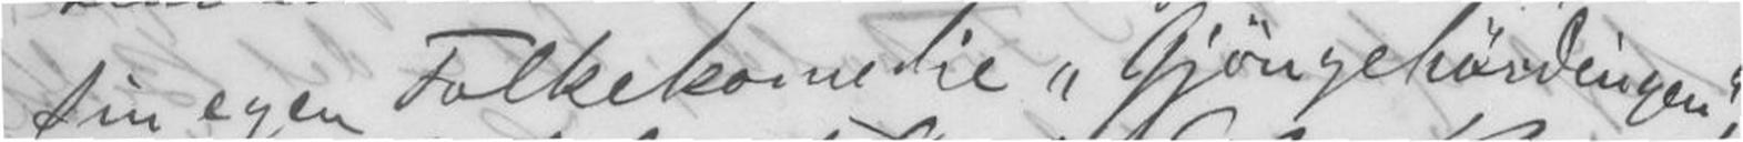sin egen Folkekomedie Gjøngehøvdingen,
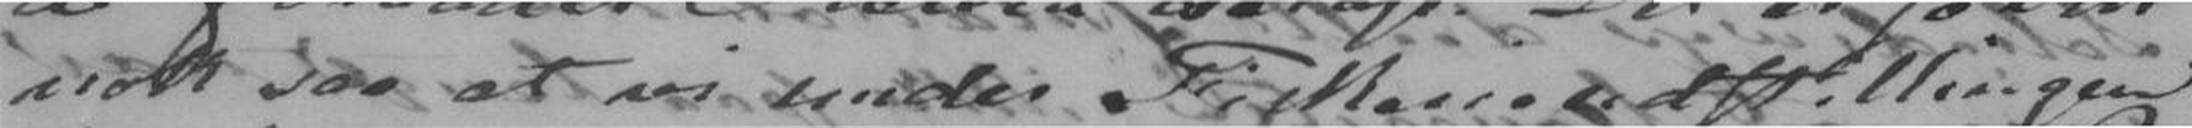nok saa at vi under Fiskerieudstillingen
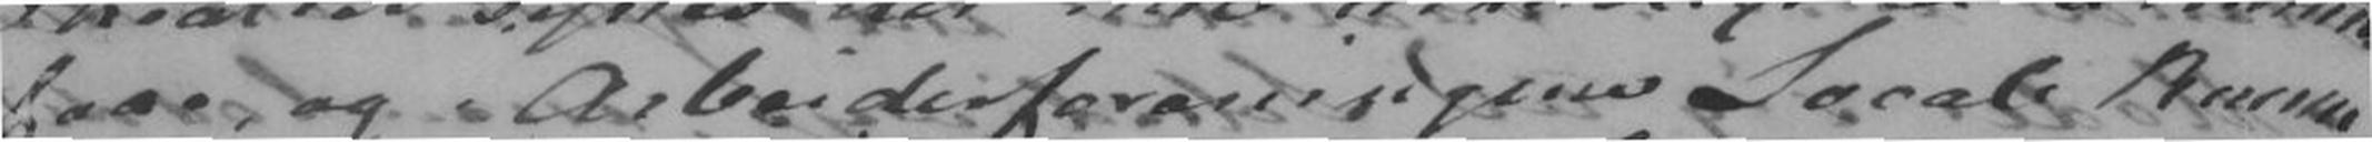faae, og Arbeiderforeningens Locale kunne
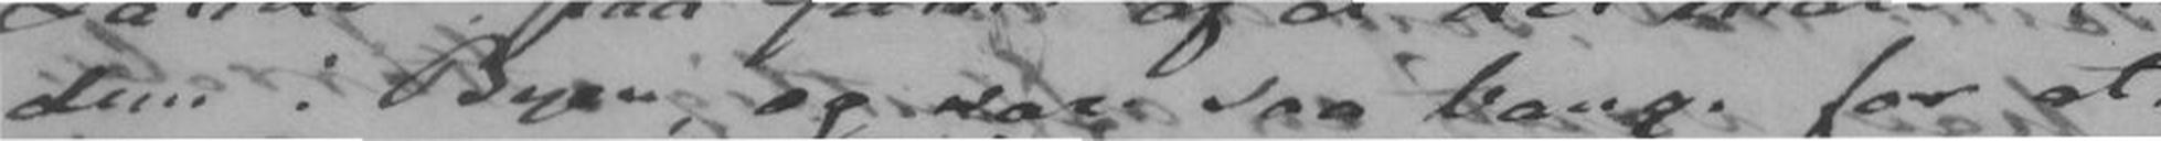dem i Byen, og vare saa bange for at
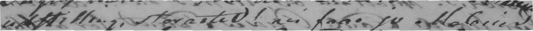udstilling, storartet! vi faae jo Malerier
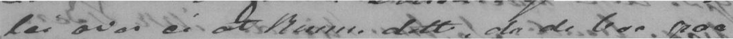lei over ei at kunne dette, da de boe paa
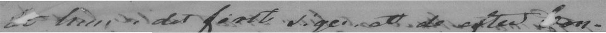at hun i det første siger at de efter Con-
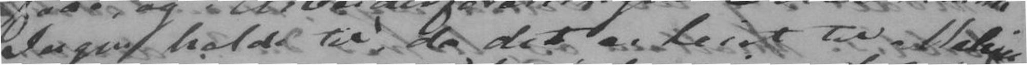Ingen holde til, da det er leiet til Maleri-
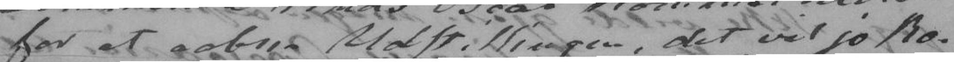for at aabne Udstilling, det vil jo ko-
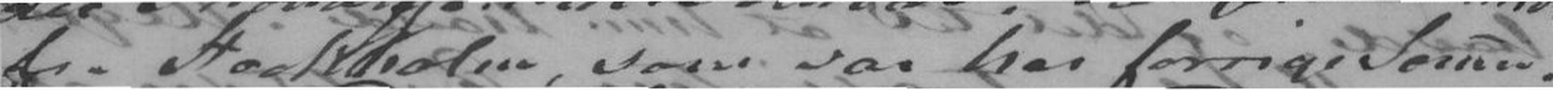fra Stockholm, som var her forrige So'mer
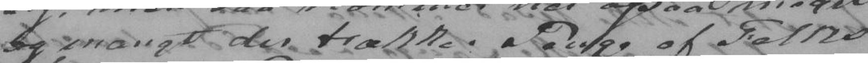og mangt der trækker Penge af Folks
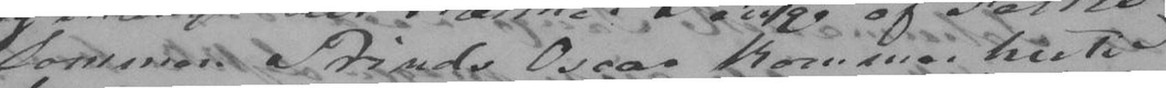Lommer. Prinds Oscar kommer hertil
